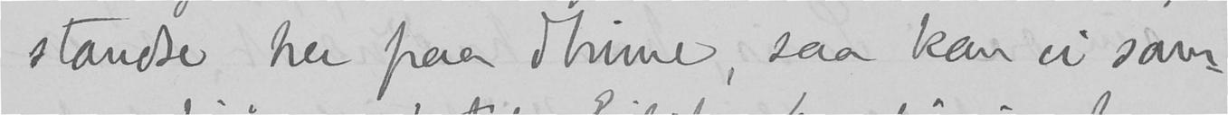standse her paa Thime, saa kan vi sam-
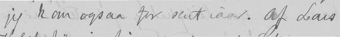jeg kom ogsaa for sent iaar. Af Lars
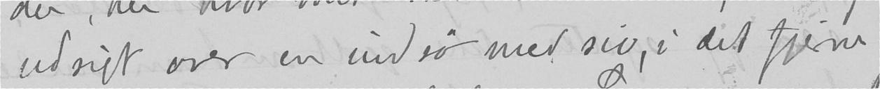udsigt over en indsø med siv, i det fjerne
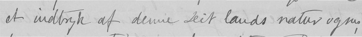et indtryk af denne Dit lands natur ogsaa,
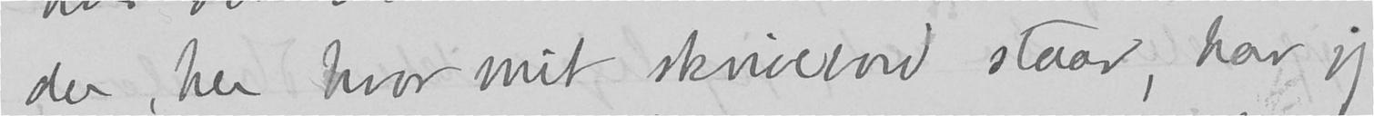du, her hvor mit skrivebord staar, har jeg
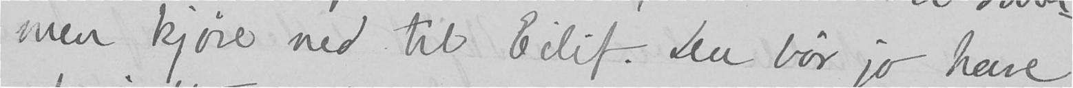men kjøre ned til Eilif. Du bør jo have
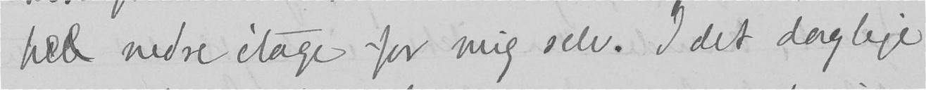hel nedre ètage for mig selv. I det daglige
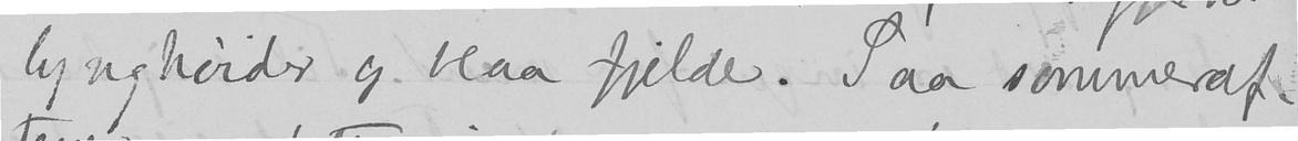lynghøider og blaa fjelde. Paa sommeraf-
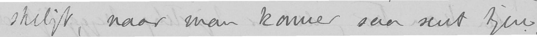skeligt, naar man kommer saa sent hjem,
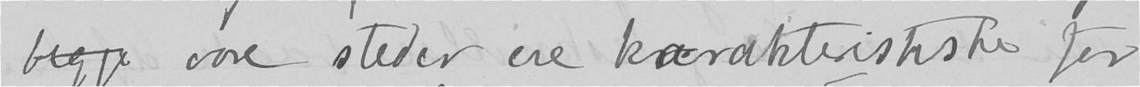begge vore steder ere karakteristiske for
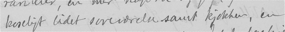koseligt lidet soveværelse samt kjøkken, en
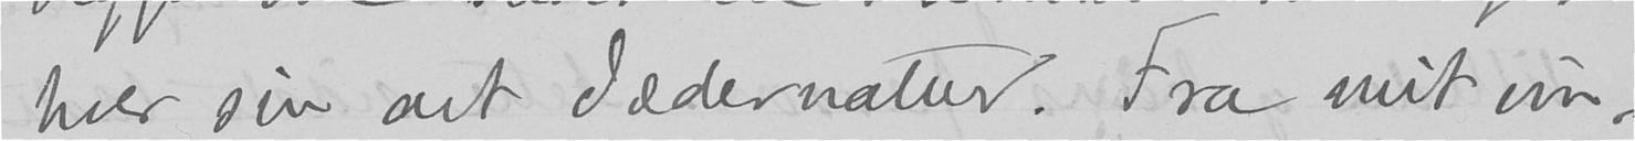hver sin art Jædernatur. Fra mit vin-
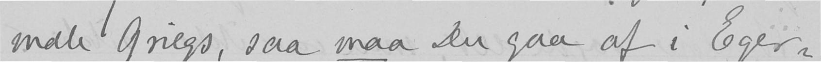male Griegs, saa maa Du gaa af i Eger-
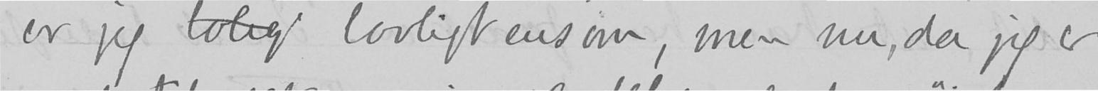er jeg lolig lovligt ensom, men nu, da jeg er
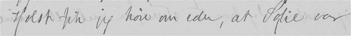Holst fik jeg høre om eder, at Sofie var
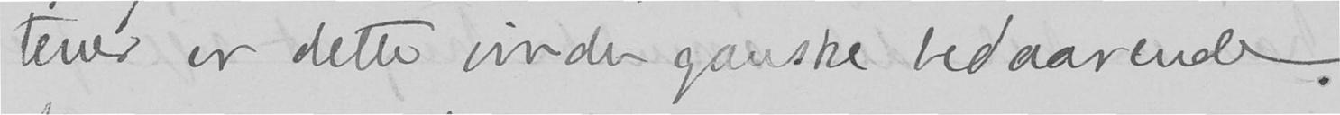tener er dette vindu ganske bedaarende.
